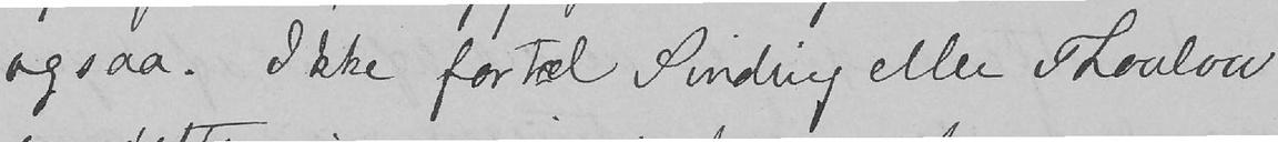ogsaa. Ikke fortæl Sinding eller Thoulow
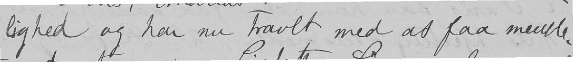lighed og har nu travlt med at faa meuble
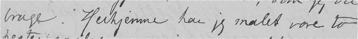bruge. Herhjemme har jeg malet vore to
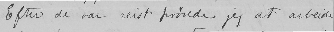Efter de var reist prøvede jeg at arbeide
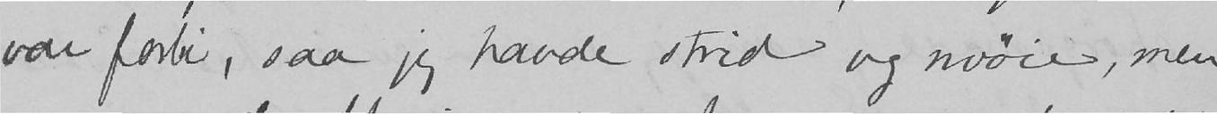var forbi, saa jeg havde strid og møie, men
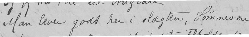Man lever godt her i slægten, Sømmes ere
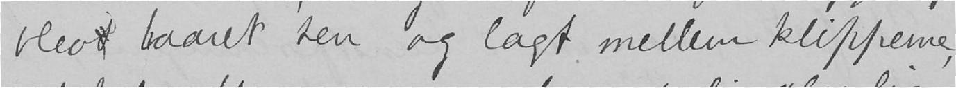blevt baaret hen og lagt mellem klipperne,
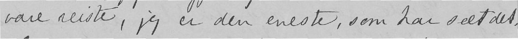vare reiste, jeg er den eneste, som har seet det.
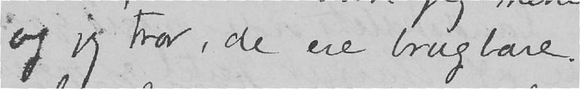og jeg tror, de ere brugbare.
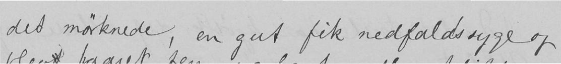det mørknede, en gut fik nedfaldssyge og
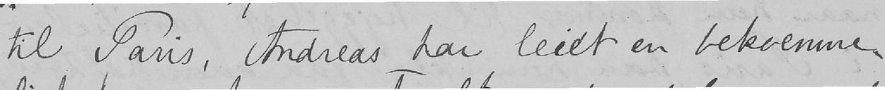til Paris, Andreas har leiet en bekvemme-
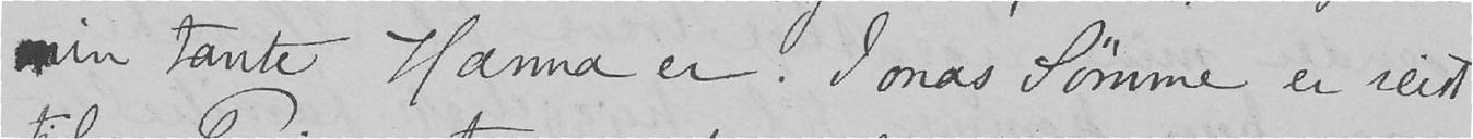min tante Hanna er. Jonas Sømme er reist
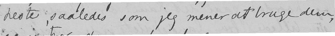heste, saaledes som jeg mener at bruge dem,
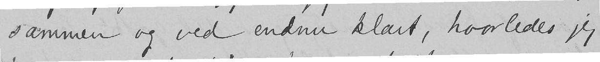sammen og ved endnu klart, hvorledes jeg
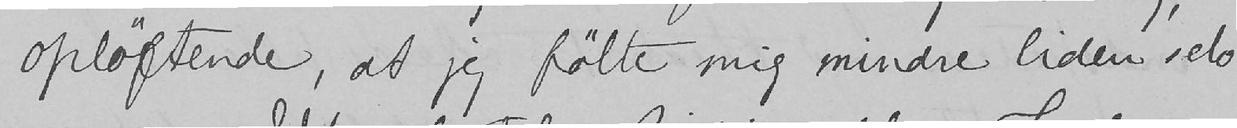opløftende, at jeg følte mig mindre liden selv
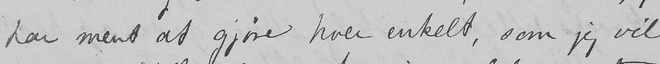har ment at gjøre hver enkelt, som jeg vil
